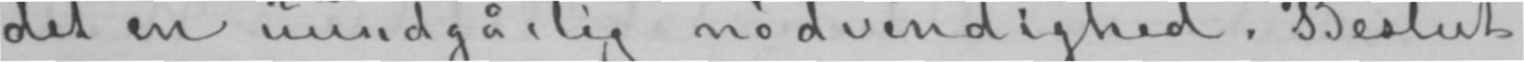det en uundgåelig nødvendighed. Beslut
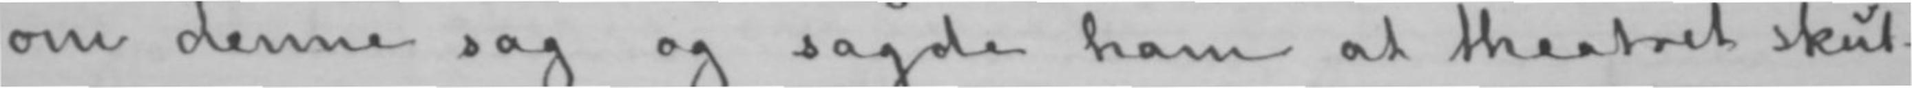om denne sag og sagde ham at theatret skul-
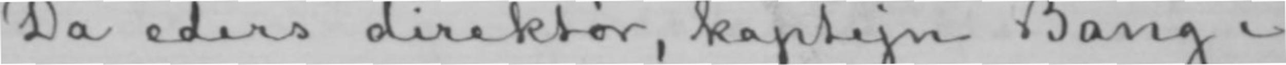Da eders direktør, kaptejn Bang i
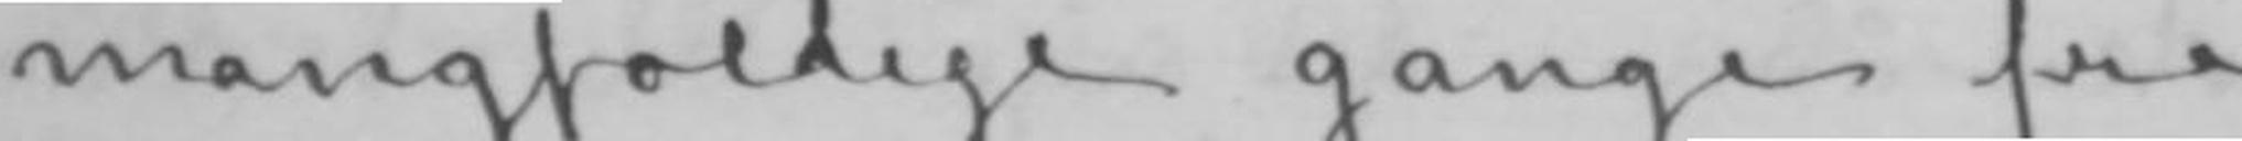mangfoldige gange fra
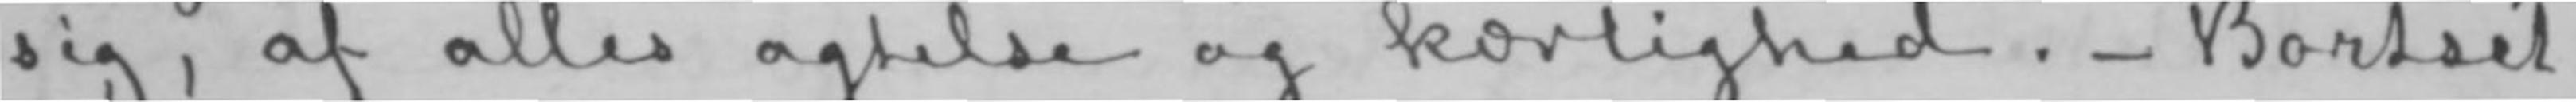sig, af alles agtelse og kærlighed.- Bortsét
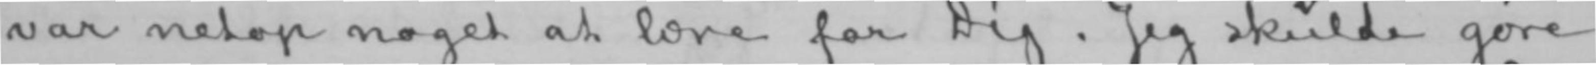var netop noget at lære for Dig. Jeg skulde gøre
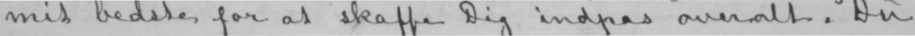mit bedste for at skaffe Dig indpas overalt. Du
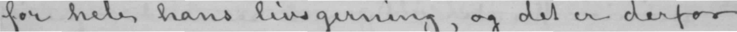for hele hans livsgerning, og det er derfor
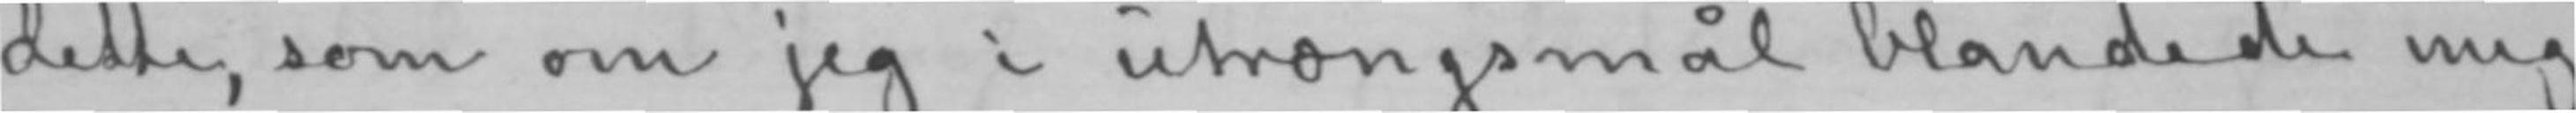dette, som om jeg i utrængsmål blandede mig
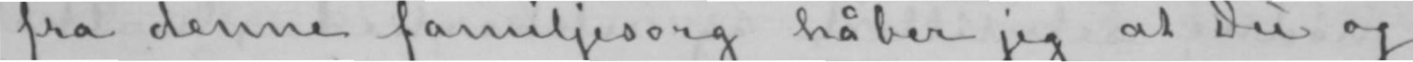fra denne familjesorg håber jeg at Du og
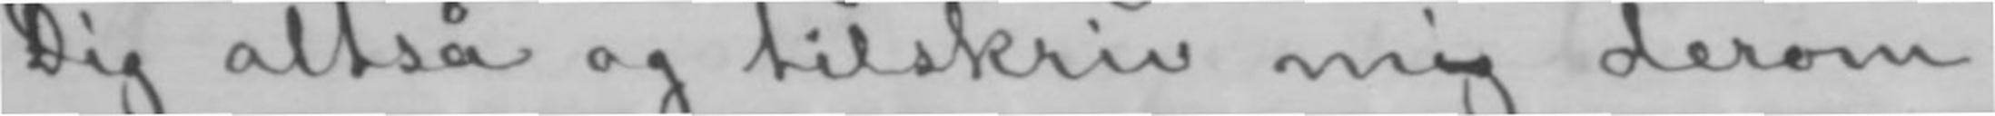Dig altså og tilskriv mig derom.-
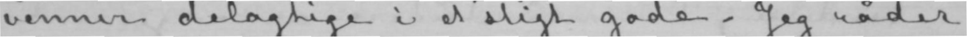venner delagtige i et sligt gode. Jeg råder
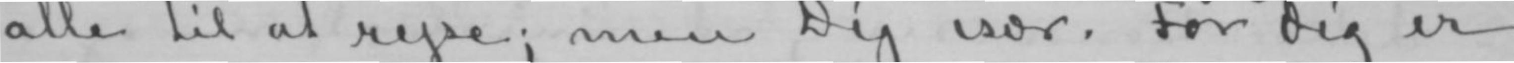alle til at rejse; men Dig især. For Dig er
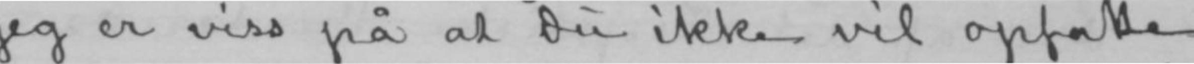Jeg er viss på at Du ikke vil opfatte
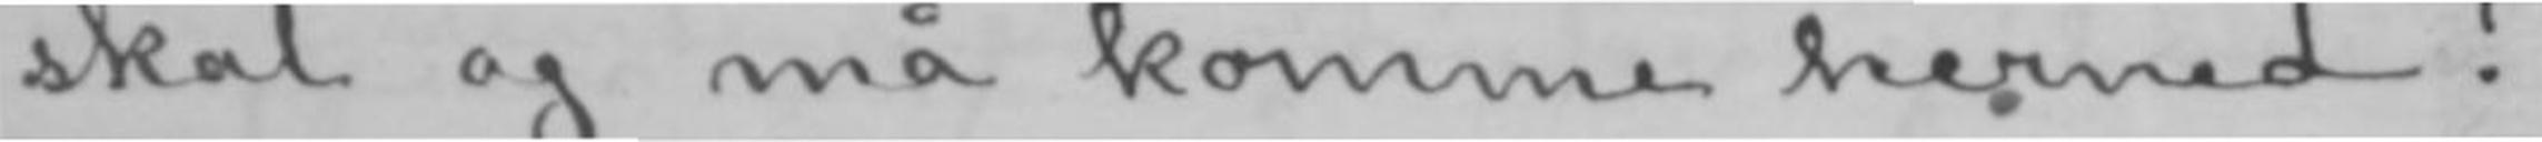skal og må komme herned!
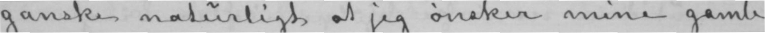ganske naturligt at jeg ønsker mine gamle
